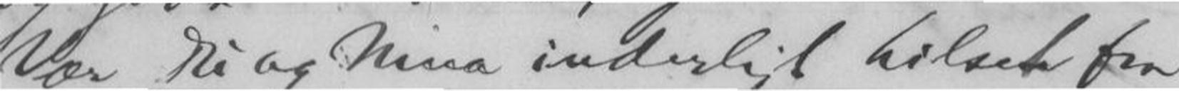Vær Du og Nina inderligt hilset fra
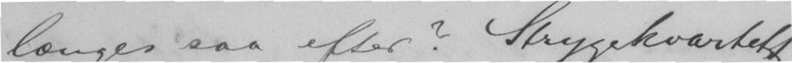længes saa efter? Strygekvartett.
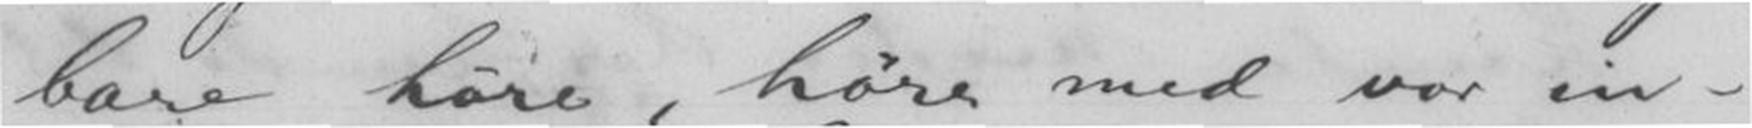bare høre, høre med vor in-
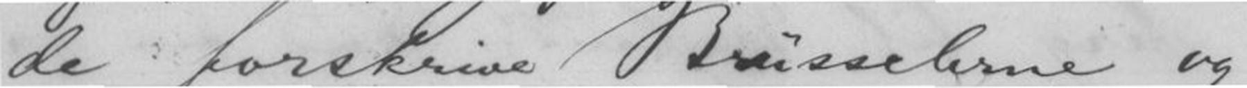de forskrive Brüsselerne og
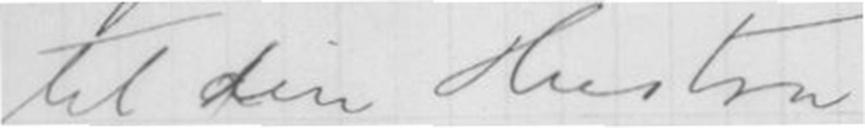til din Hustru
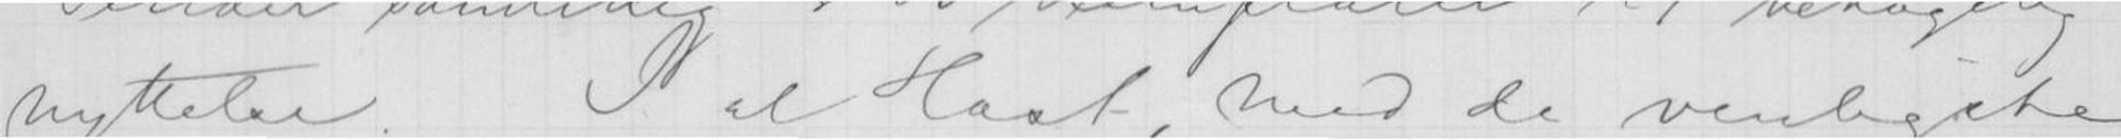nyttelse. I al Hast, med de venligste
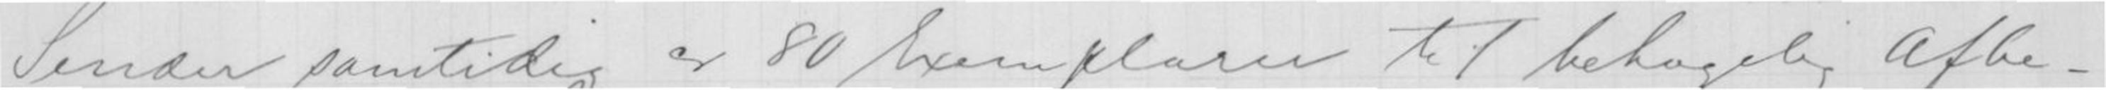Sender samtidig cr 80 Exemplarer til behagelig Afbe-
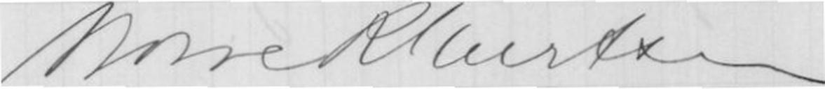Børre R Giertsen
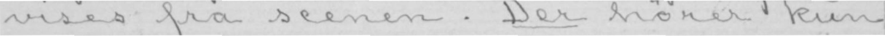vises fra scenen. Der hører kun
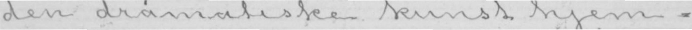den dramatiske kunst hjem-
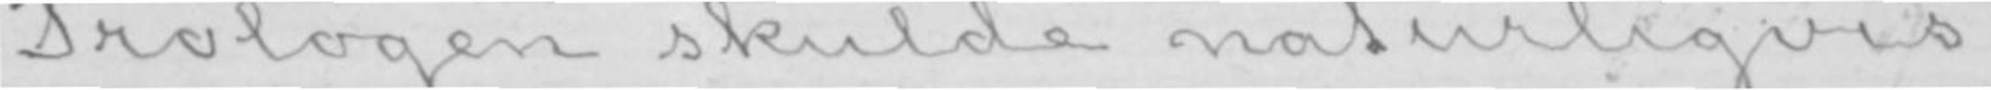Prologen skulde naturligvis
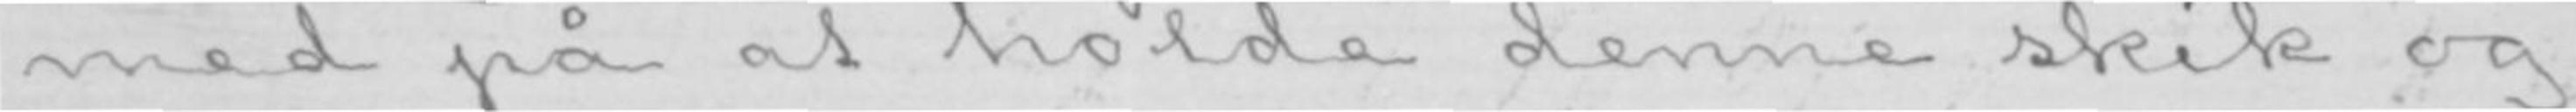med på at holde denne skik og
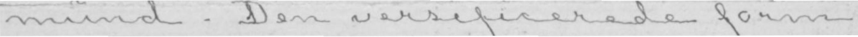mund. Den versificerede form
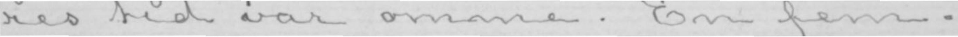res tid var omme. En fem-
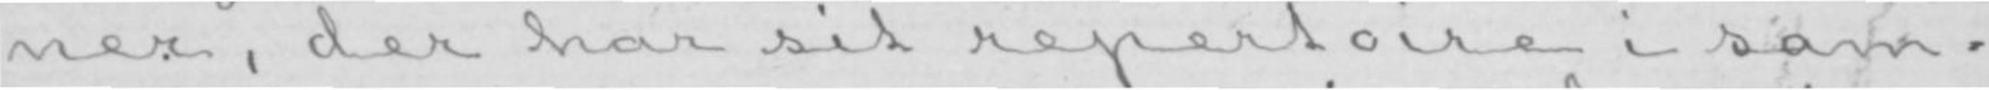ner, der har sit repertoire i sam-
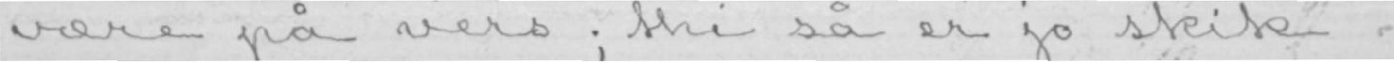være på vers; thi så er jo skik
